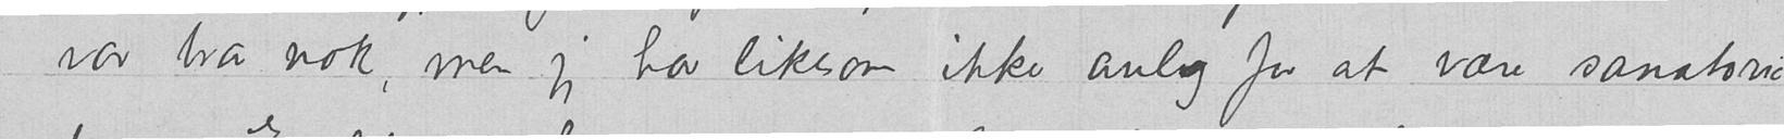var bra nok, men jeg har likesom ikke anlæg for at være sanatori-
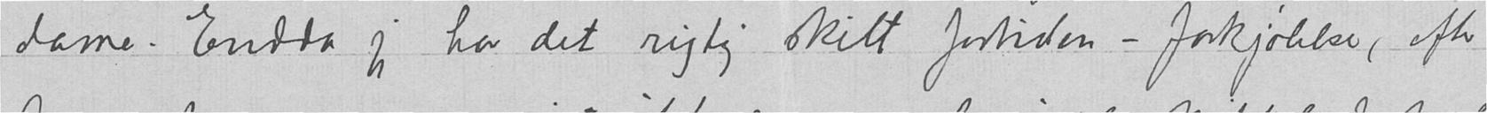dame. Endda jeg har det rigtig skitt fortiden – forkjølelse (efter
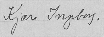Kjære Ingeborg,
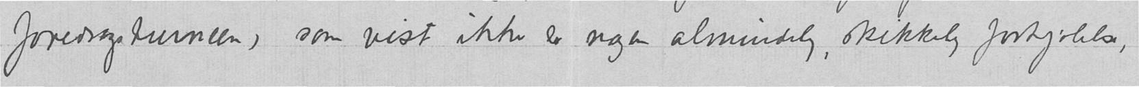foredragsturneen) som vist ikke er nogen almindelig, skikkelig forkjølelse,
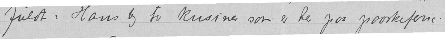fuldt: Hans og to kusiner som er her paa paaskeferie.
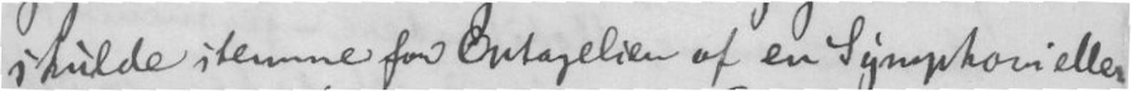skulde stemme for Optagelsen af en Synphoni eller
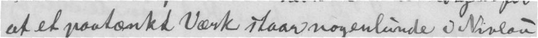at et paatænkt Værk staar nogenlunde i Niveou
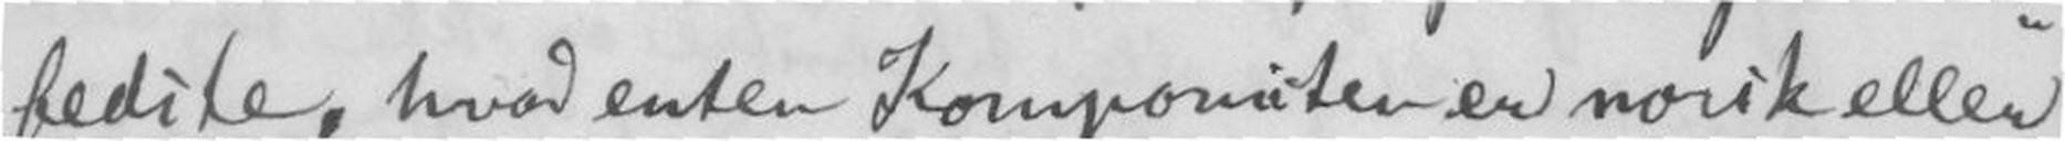bedste, hvad enten Komponisten er norsk eller
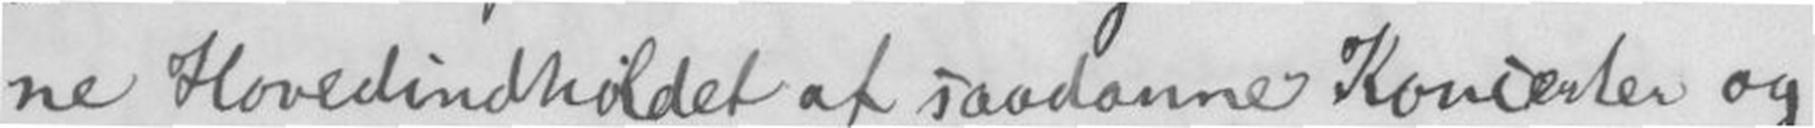ne Hovedindholdet af saadanne Koncerter og
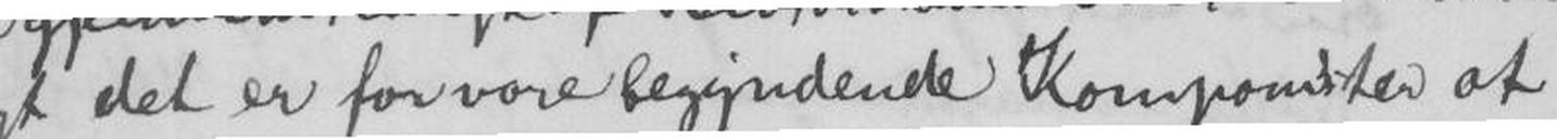digt det er for vore begyndende Komponister at
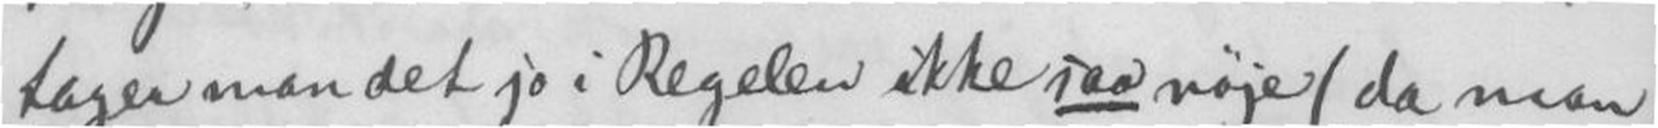tager man det jo i Regelen ikkesaa nøje (da man
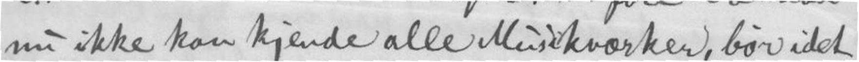nu ikke kan kjende alle Musikværker, bør idet-
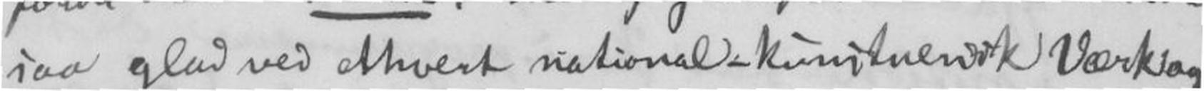saa glad ved ethvert national-kunstnerisk Værk og
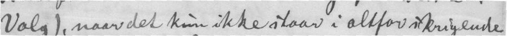Valg), naar det kun ikke staar i altfor skrigende
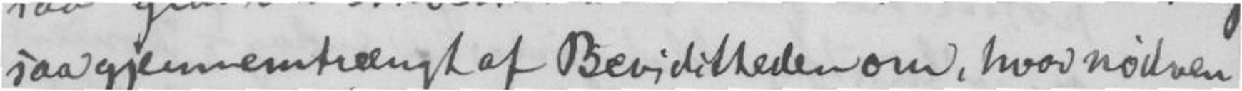saa gjennemtrængt af Beviditheden om, hvor nødven-
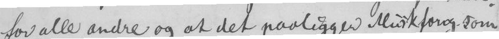for alle andre og at det paaligger Musikforrg som
